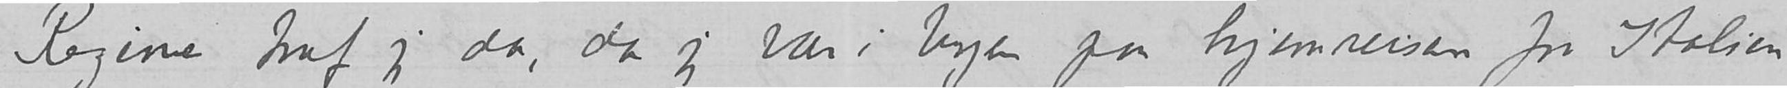Regine traf jeg da, da jeg var i byen paa hjemveien fra Italien.
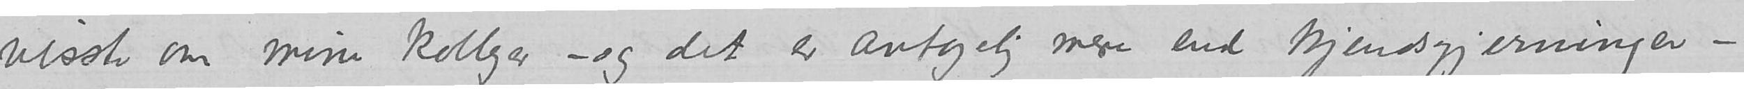visste om mine kolleger – og det er antagelig mere end kjendsgjerninger –.
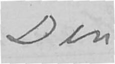Din
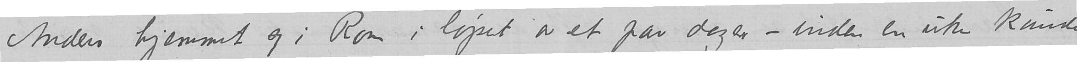Anders hjemmet sig i Rom i løpet av et par dager – inden en uke kunde
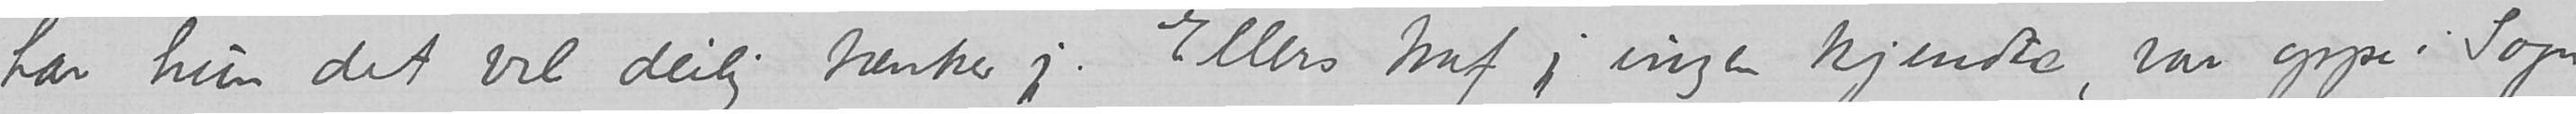har hun det vel deilig tænker jeg. Ellers traf jeg ingen kjendte, var oppe i Sogn
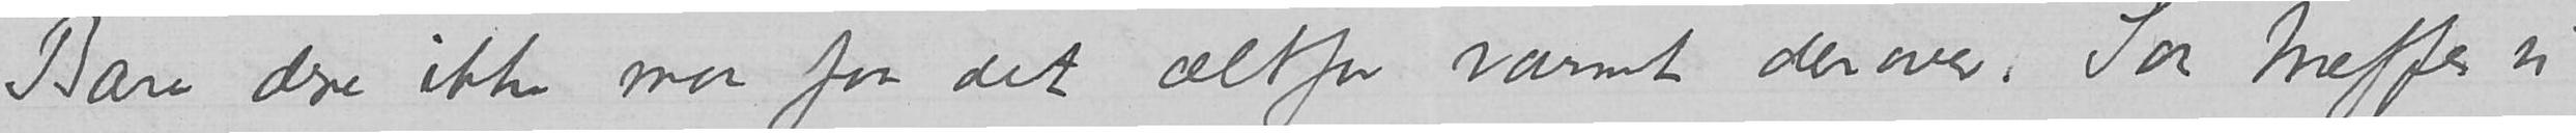Bare dere ikke maa faa det altfor varmt derover. Saa treffes vi
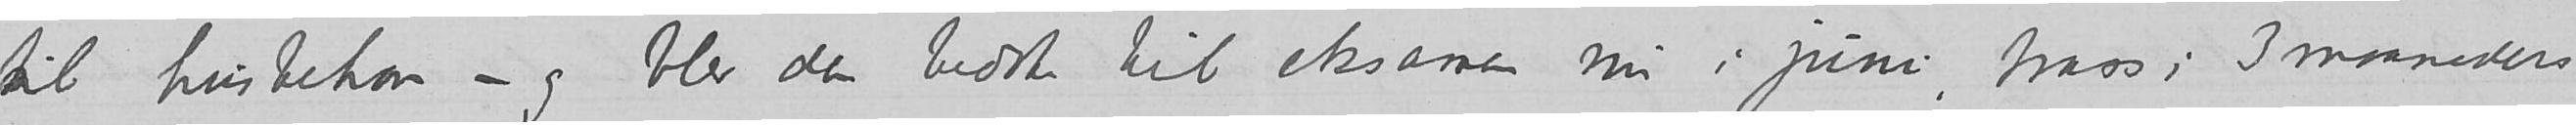til husbehov – og ble den bedste til eksamen nu i juni, trass i 3 maaneders
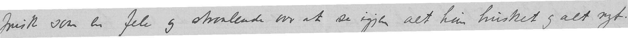frisk som en fele og straalende over at se igjen alt hun husket og alt nyt.
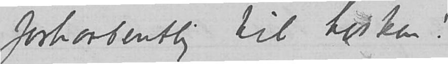forhaabentlig til høsten!
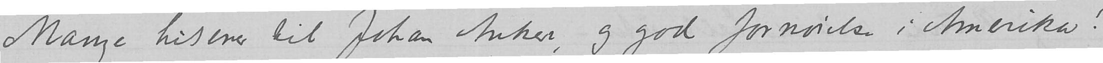Mange hilsener til Johan Anker, og god fornøielse i Amerika!
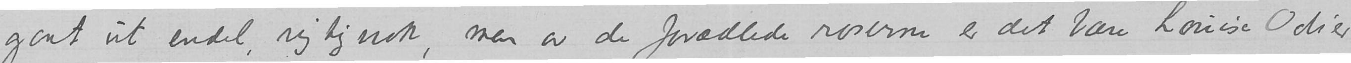gaat ut en del, rigtignok, men av de forædlede roserne er det bare Louise
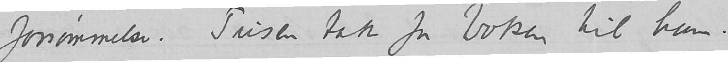forsømmelse. Tusen tak for boken til ham!
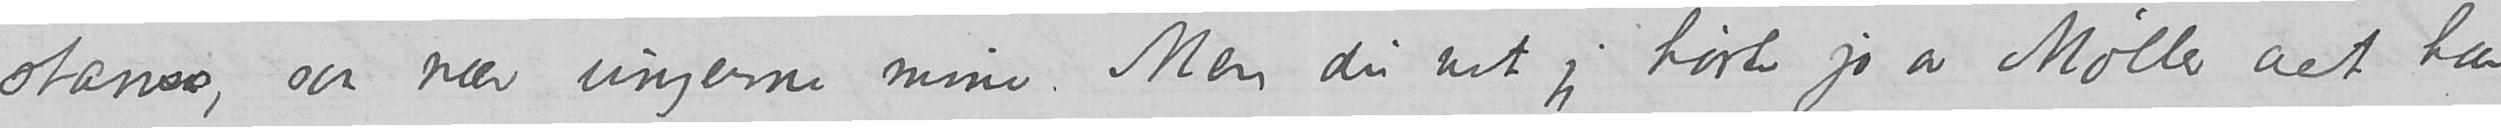stanse, saa nær ungerne mine. Men du vet jeg hørte jo av Møller alt han
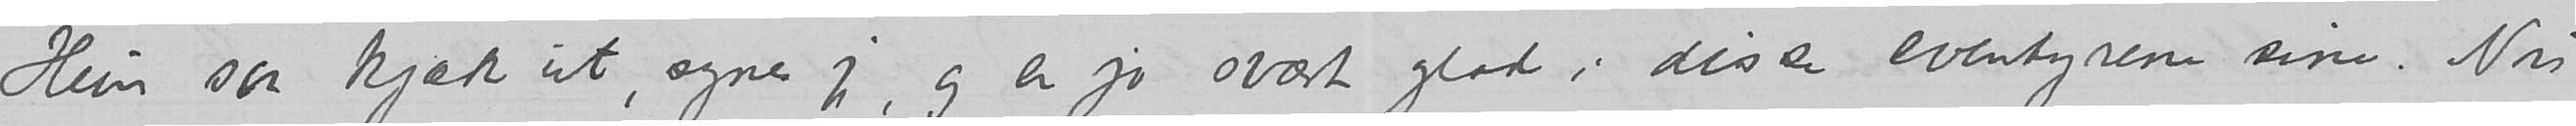Hun saa kjæk ut, synes jeg, og er jo svært glad i disse eventyrene sine. Nu
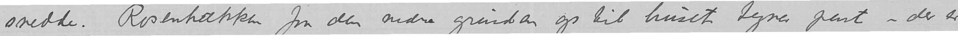snedde. Rosenhækken fra den nedre grinden op til huset tegner pent – der er
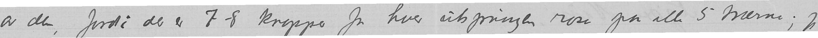av den, fordi der er 7-8 knopper for hver utsprungen rose paa alle 5 trærne; jeg
